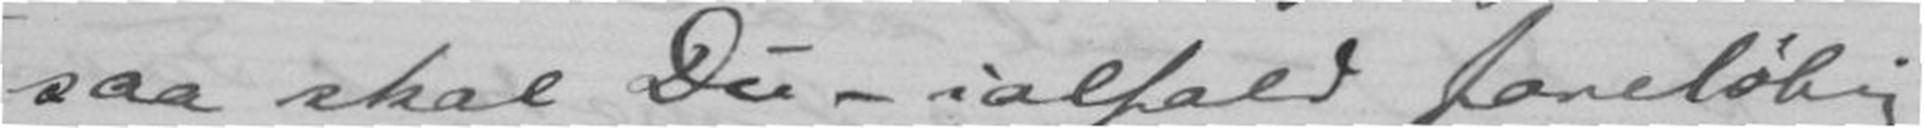saa skal Du - ialfald foreløbig
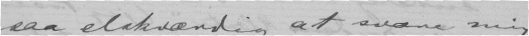saa elskværdig at svare mig
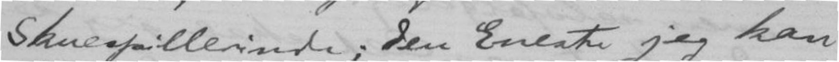skuespillerinde; den Eneste jeg kan
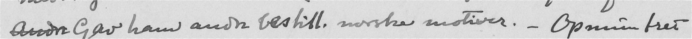Andre Gav ham andre bestill. norske motiver. - Opmuntret
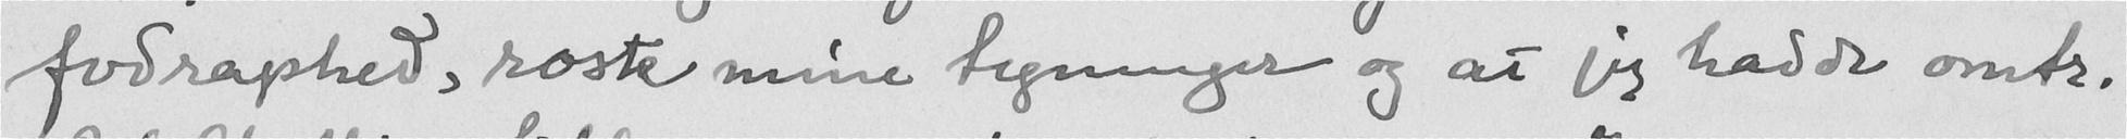fodraphed, roste mine tegninger og at jeg hadde omtr.
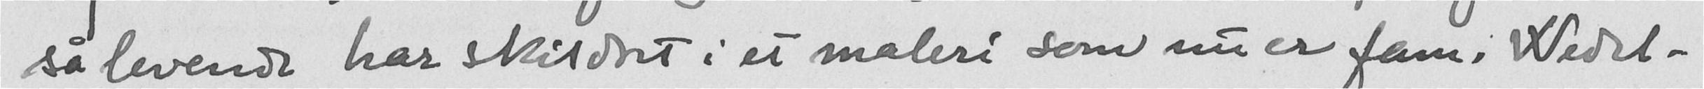så levende har skildret i et maleri som nu er fam. Wedel-
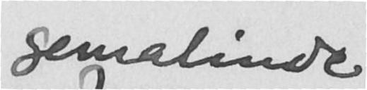gemalinde
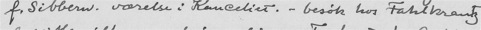f. Sibbern. værelse i Kanceliet. - besøk hos Fahlkrantz
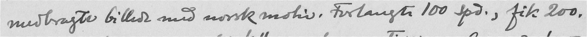medbragte billede med norsk motiv. Forlangte 100 spd., fik 200.
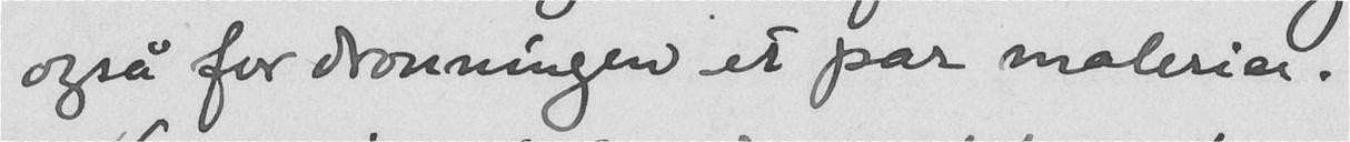også for dronningen et par malerier.
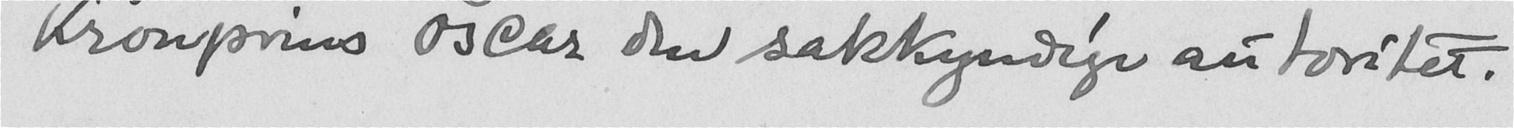Kronprins Oscar den sakkyndige autoritet.
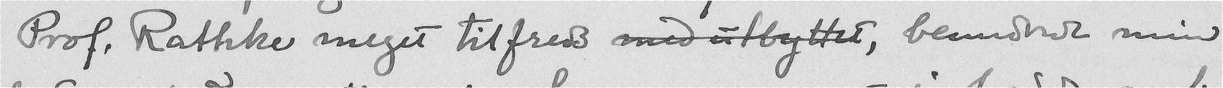Prof. Rathke meget tilfreds med utbyttet, beundrede min
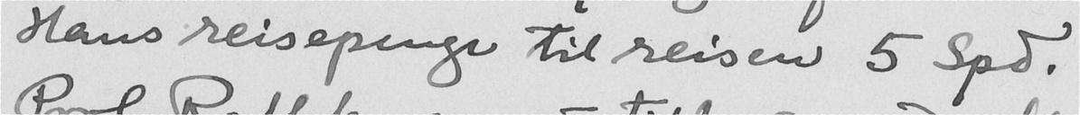Hans reisepenge til reisen 5 spd.
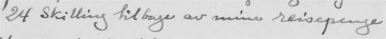24 Skilling tilbage av mine reisepenge
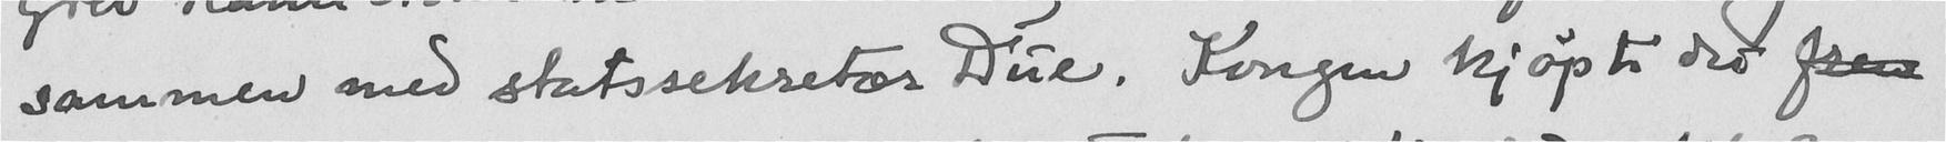sammen med statssekretær Due. Kongen kjøpte det fra
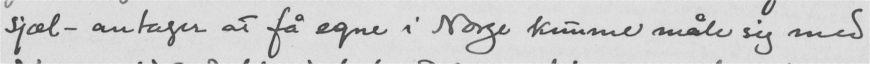sjæl - antager at få egne i Norge kunne måle sig med
